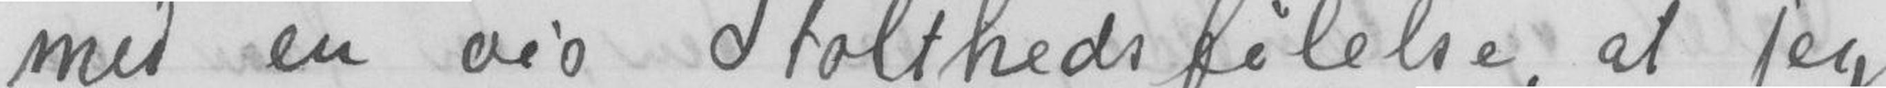med en vis Stolthedsfølerse, at jeg
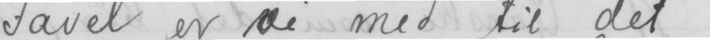Javel er De med til det
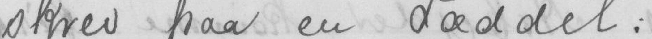skrev paa en Sæddel.
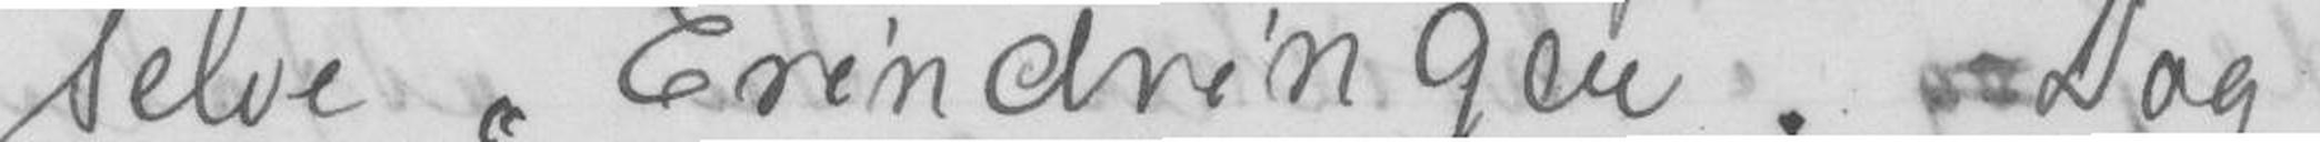selve "Erindringen" Dag Dog
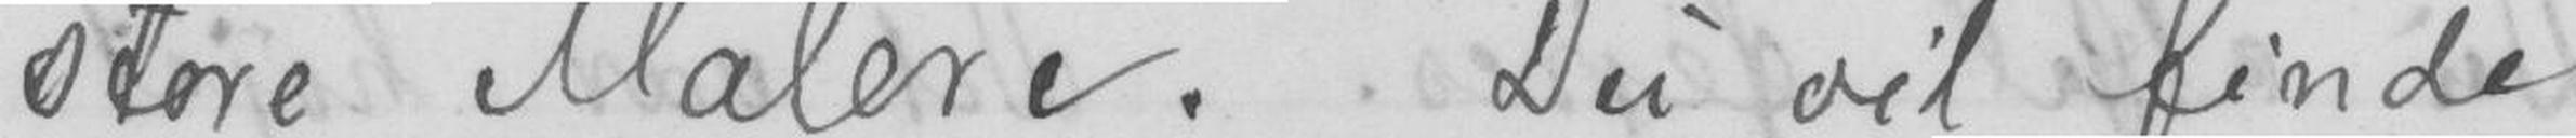store Maleri. Du vil finde
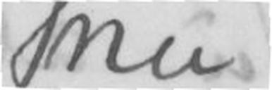nu
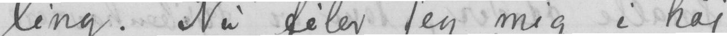ling. Nu føler jeg mig i høi
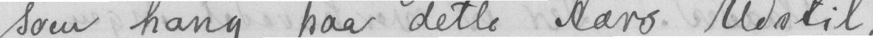som hang paa dette Aars Udstil-
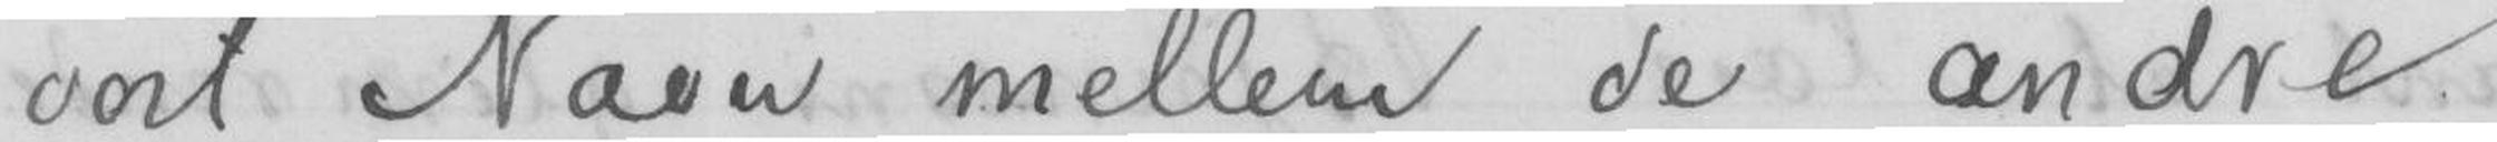vort Navn mellem de andre
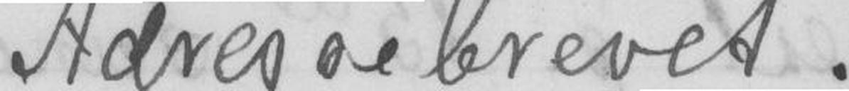Adressebrevet.
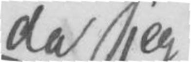da jeg
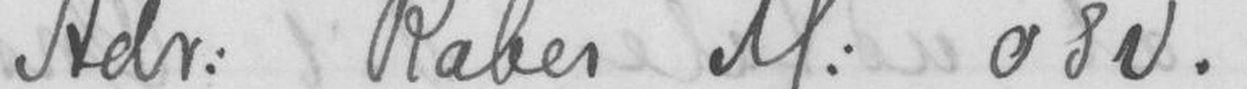Adr. Rabes M. osv.-
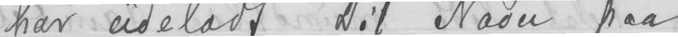har udeladt Dit Navn paa
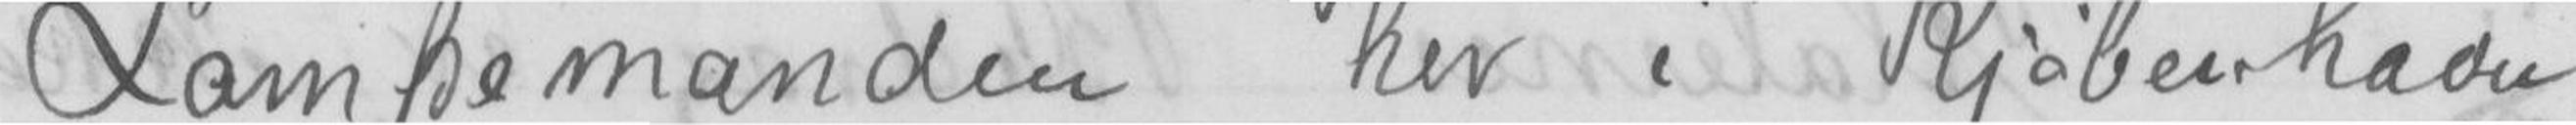Lampemanden her i Kjøbenhavn
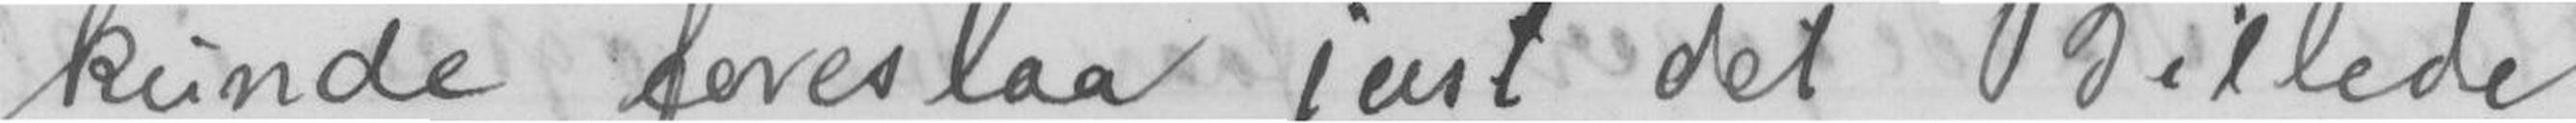kunde foreslaa just det Billede,
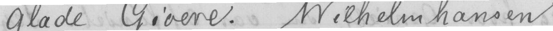glade Givere. Wilhelm Hansen
